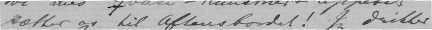sætter os til Aftensbordet! Jeg drikker
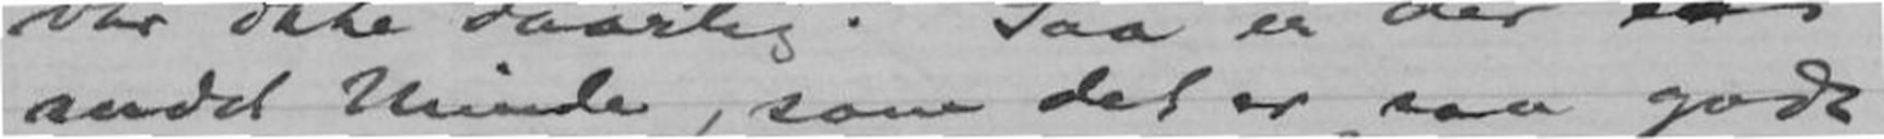andet Minde, som det er saa godt
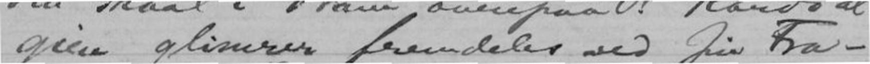gien glimrer fremdeles ved sin Fra-
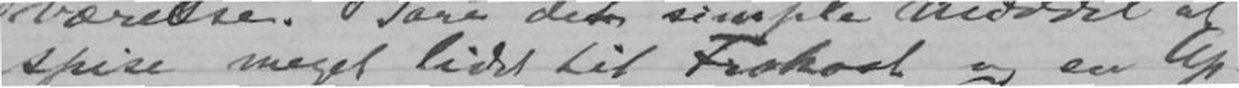spise meget lidet til Frokost og en Ap-
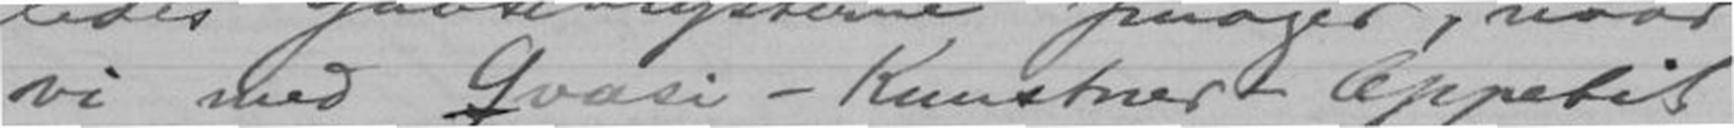vi med Qvasi-Kunstner-Appetit
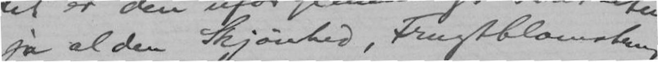ja al den Skjønhed, Frugtblomstringen,
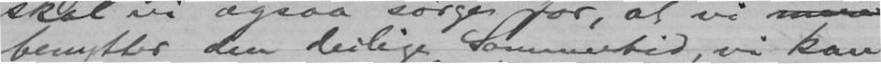benytter den deilige Sommertid, vi kan
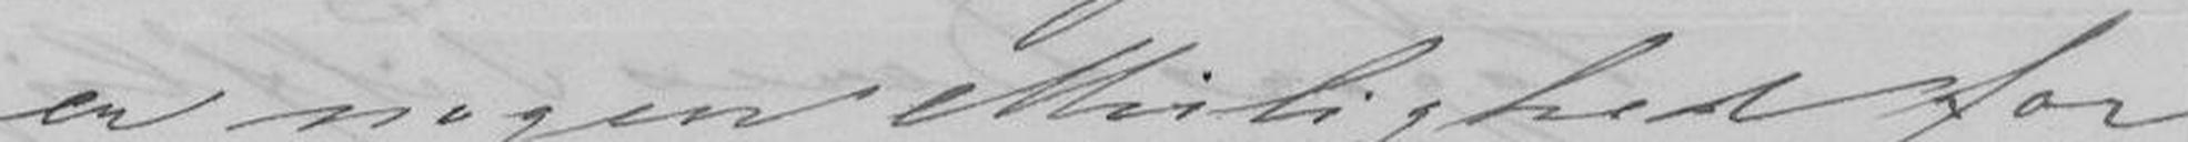er nogen Mulighed for
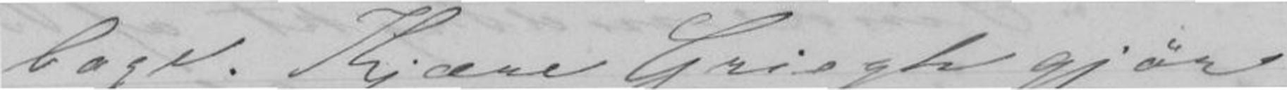bage. Kjære Griegh gjør
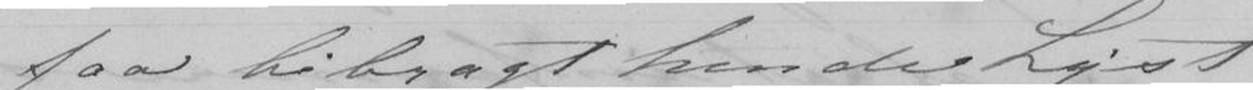faa bibragt hendes Lyst
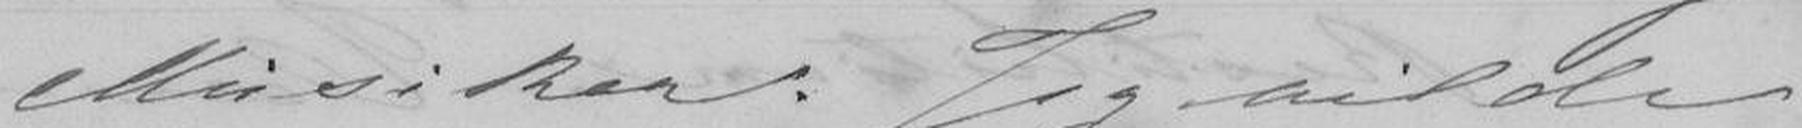Musiker. Jeg vilde
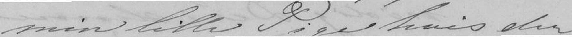min lille Pige hvis der
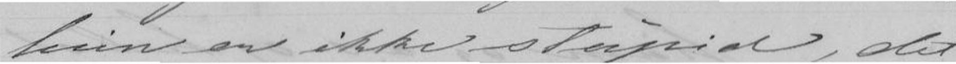hun er ikke stupid, det
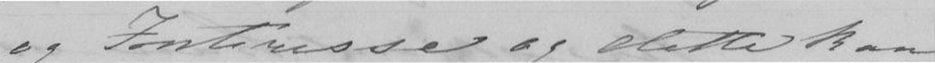og Interesse og dette kan
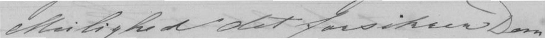Mulighed det forsikrer Dem
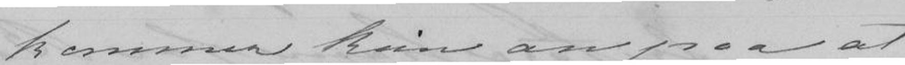kommer kun anpaa at
